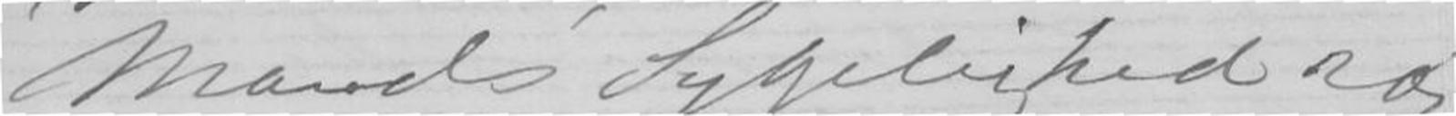Mands Sygelighed røre
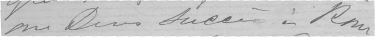om Deres Succes i Rom
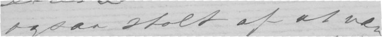ogsaa stolt af at være
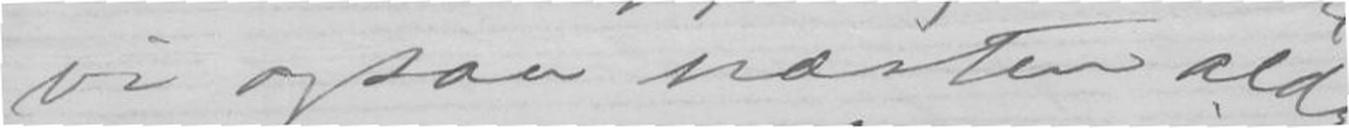vi ogsaa næsten aldrig
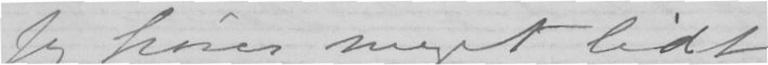Jeg hører meget lidt
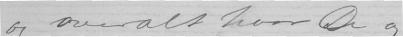og overalt hvor De og
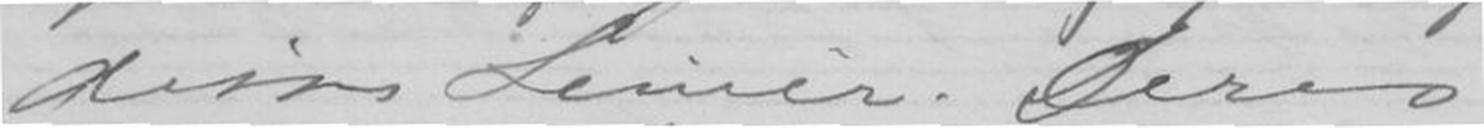disse linier. Deres
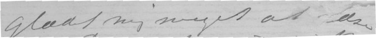glædet mig meget at æse
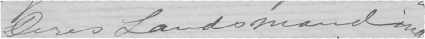Deres Landsmandinde.
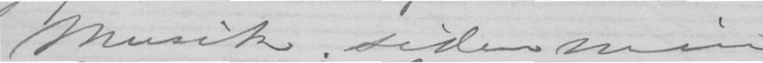Musik; siden min
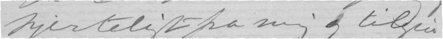hjerteligt fra mig og tilgive
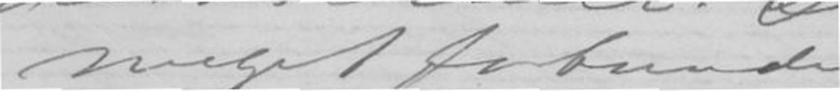meget forbunden
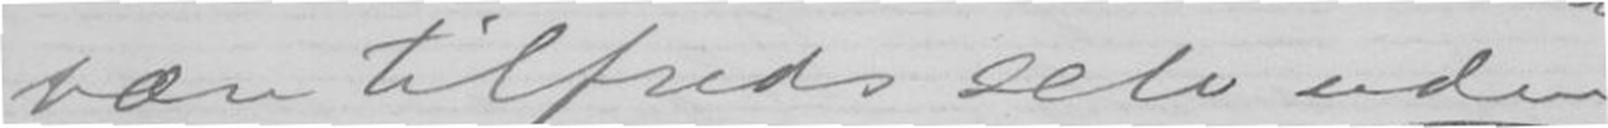være tilfreds selv uden
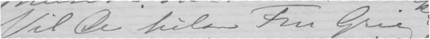Vil De hilse Fru Grieg
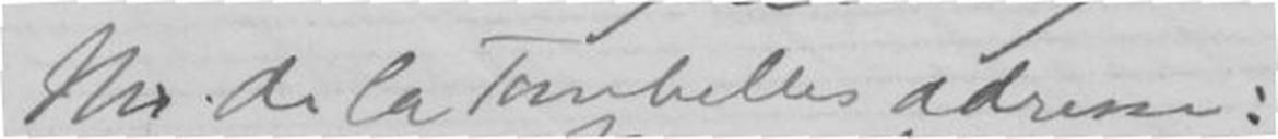Mr de la Tombelles adresse:
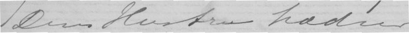Deres Hustru hædrer
